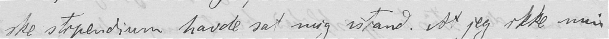ske stipendium havde sat mig istand. At jeg ikke min
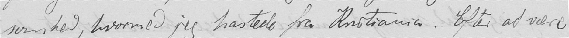somhed, hvormed jeg hastede fra Kristiania. Efter at være
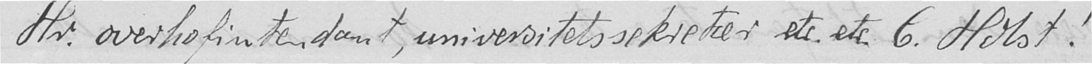Hr. overhofintendant, universitetssekretær etc. etc. C. Holst!
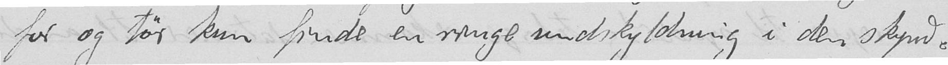for og tør kun finde en ringe undskyldning i den skynd-
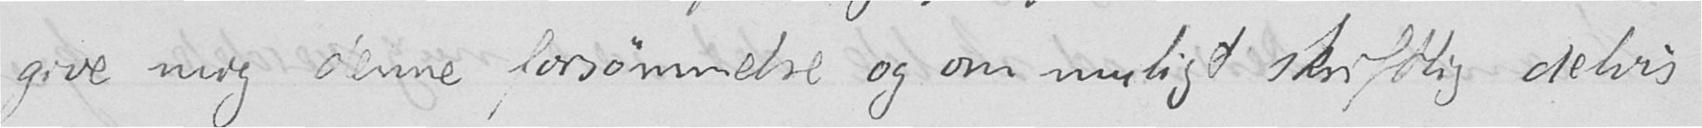give mig denne forsømmelse og om muligt skriftlig delvis
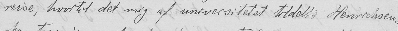reise, hvortil det mig af universitetet tildelte Henrichsen-
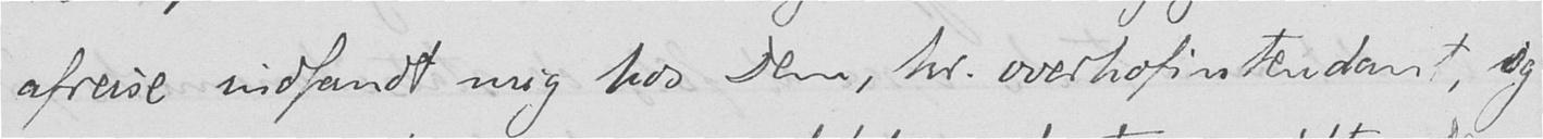afreise indfandt mig hos Dem, hr. overhofintendant, og
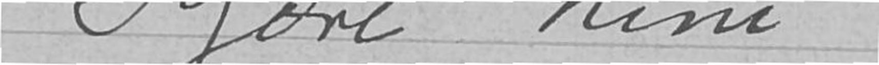Kjære Nini,
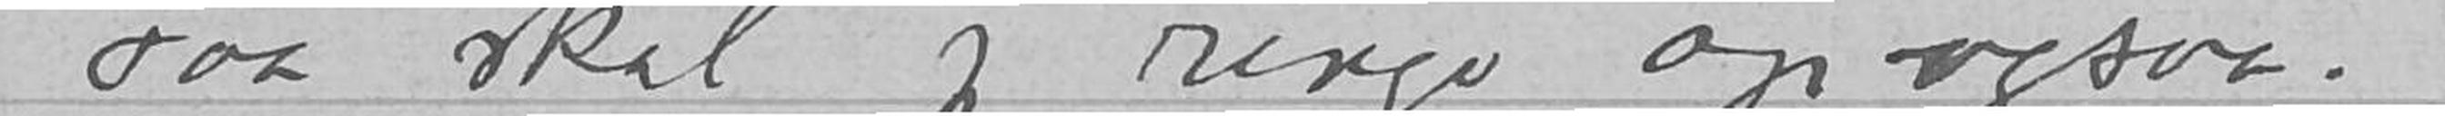saa skal jeg ringe op ogsaa
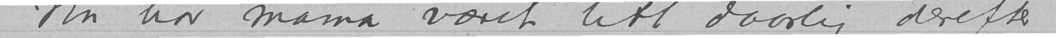Nu har mama været litt daarlig, derefter
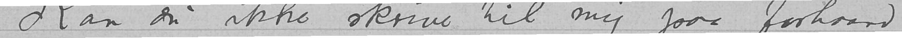Kan du ikke skrive til mig paa forhaand
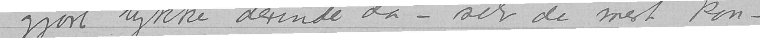gjort lykke derinde da - selv de mest kon-
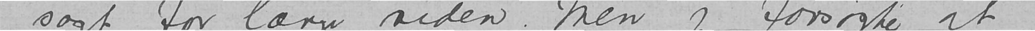sagt for længe siden. Men jeg forsøgte at
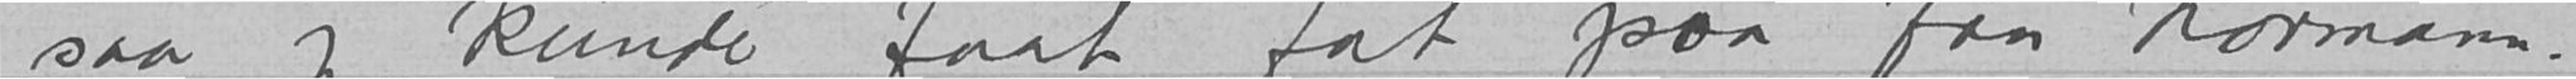saa jeg kunde faat fat paa fru Normann.
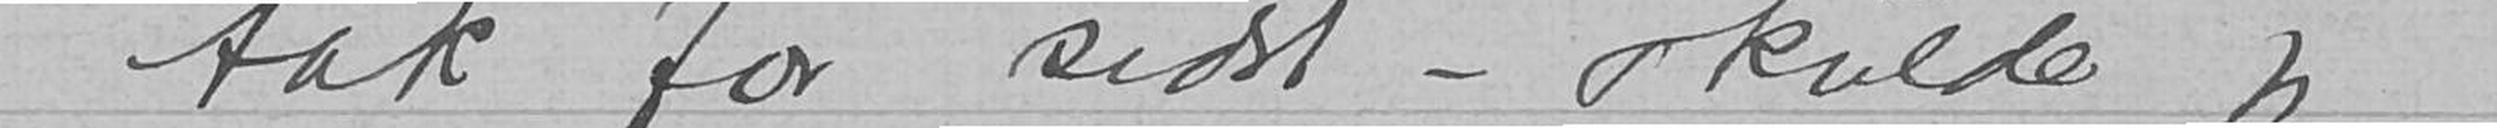tak for sidst – skulde jeg
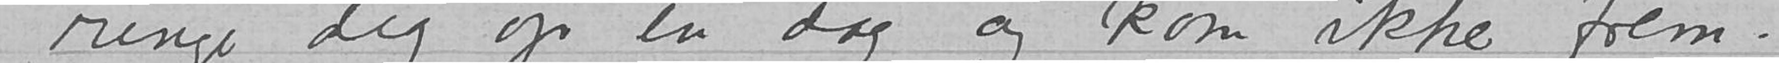ringe dig op en dag og kom ikke frem.
